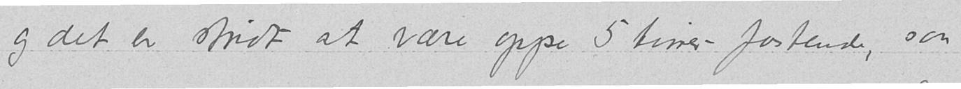og det er stridt at være oppe 5 timer – fastende, saa
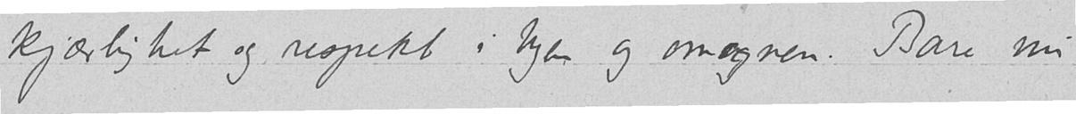kjærlighet og respekt i byen og omegnen. Bare nu
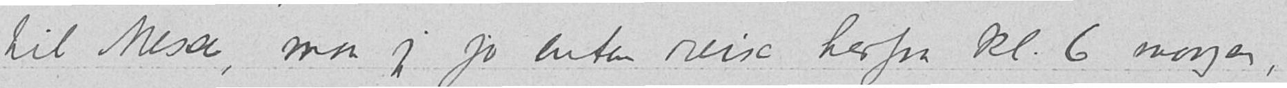til Messe, maa jeg jo enten reise herfra kl. 6 morgen,
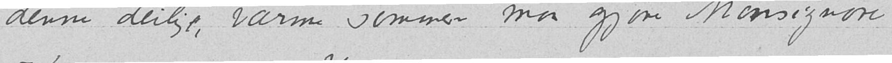denne deilige, varme sommer maa gjøre Monsignore
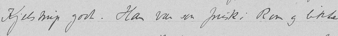Kjelstrup godt. Han var saa frisk i Rom og lekte
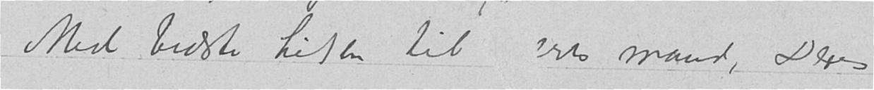Med bedste hilsen til Deres mand. Deres
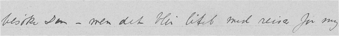besøke Dem – men det blir litet med reiser før mig
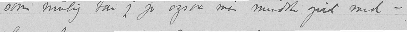som mulig tar jeg jo ogsaa min mindste gut med –
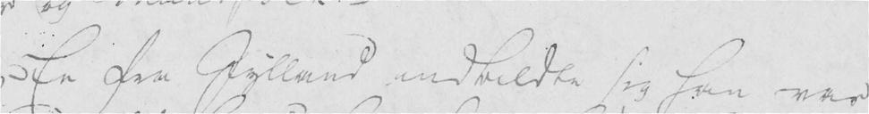En fra Jylland indbildte sig han var
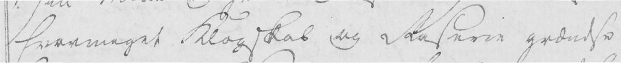hvormeget Klogskab og Sløserie grændse
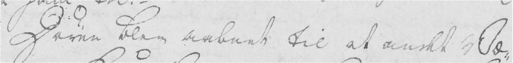Døren blev aabnet til et andet Væ-
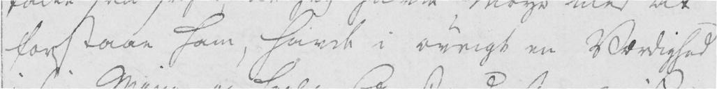forstaae ham, havde i øvrigt en Værdighed
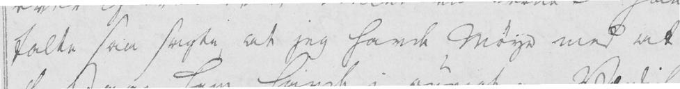talte saa sagte at jeg havde møye med at
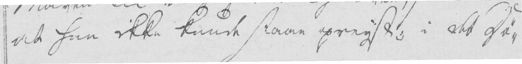at han ikke kunde staae opreyst; i det Dø-
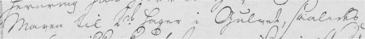Maven til 2de hager i Gulvet, saaledes
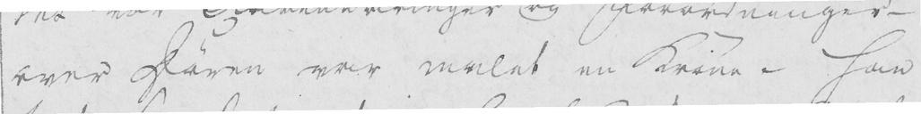over Døren var malet en Krone - han
